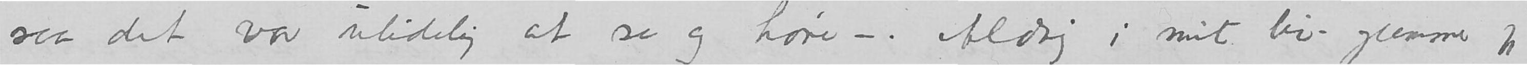saa det var ulidelig at se og høre –. Aldrig i mit liv glemmer jeg
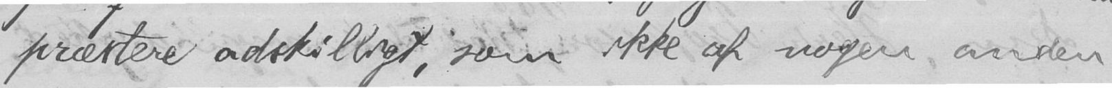præstere adskilligt, som ikke af nogen anden
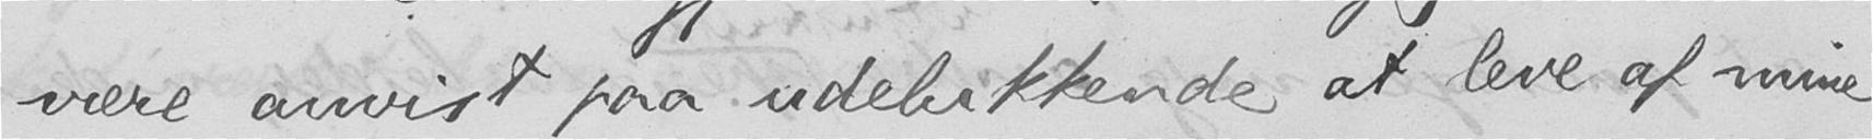være anvist paa udelukkende at leve af mine
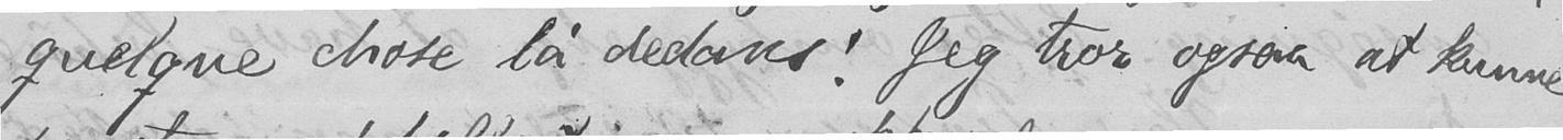quelque chose là dedans! Jeg tror ogsaa at kunne
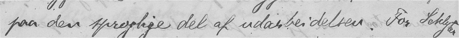paa den sproglige del af udarbeidelsen. For Schlyter
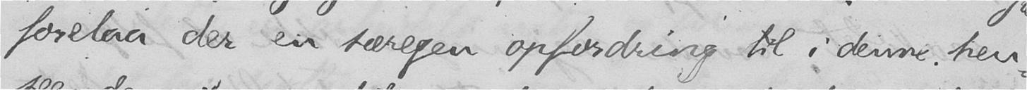forelaa der en særegen opfordring til i denne hen-
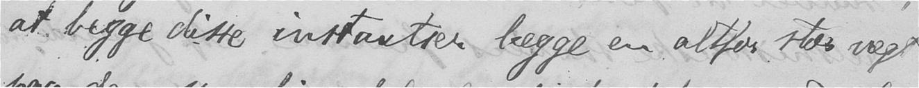at begge disse instantser lægge en altfor stor vægt
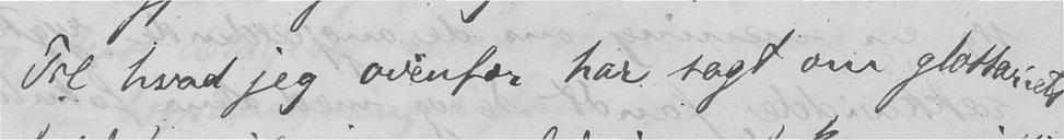Til hvad jeg ovenfor har sagt om glossariets
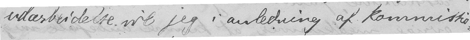udarbeidelse vil jeg i anledning af kommissio-
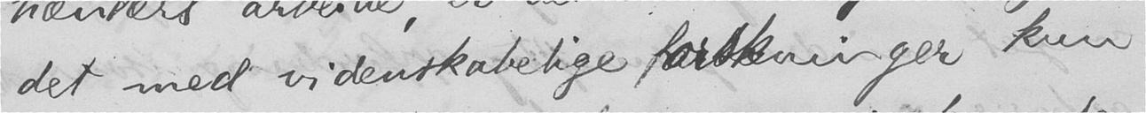det med videnskabelige forskninger kun
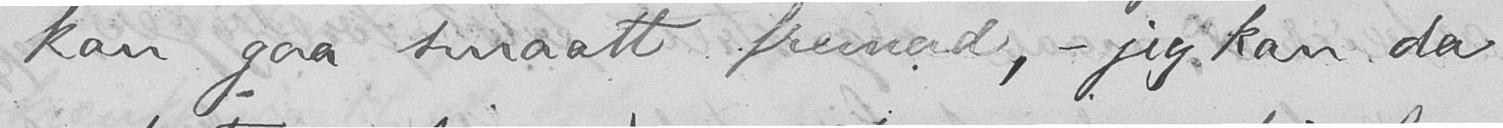kan gaa smaatt fremad, – jeg kan da
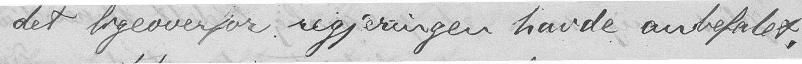det ligeoverfor regjeringen havde anbefalet,
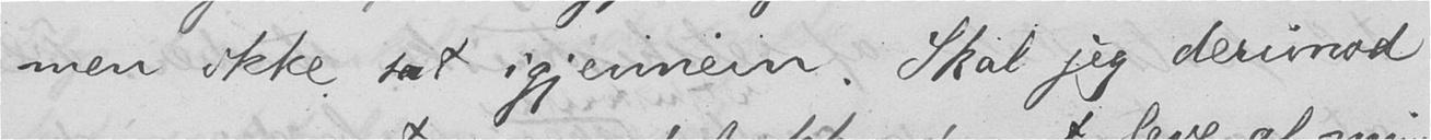men ikke sat igjennem. Skal jeg derimod
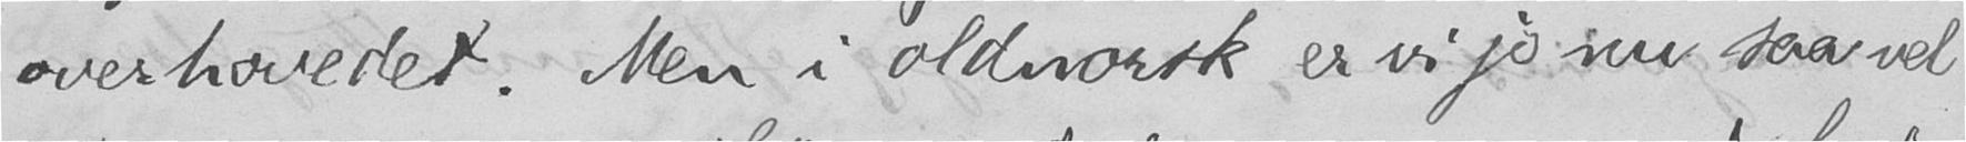overhovedet. Men i oldnorsk er vi jo nu saa vel
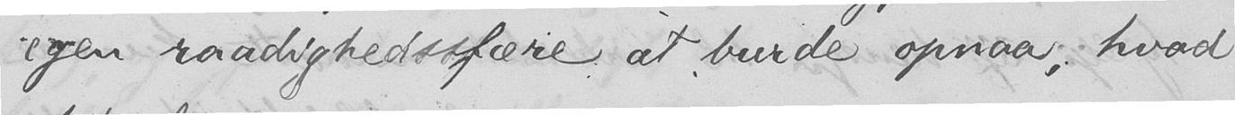egen raadighedssfære at burde opnaa, hvad
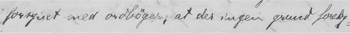forsynet med ordbøger, at der ingen grund forelig-
